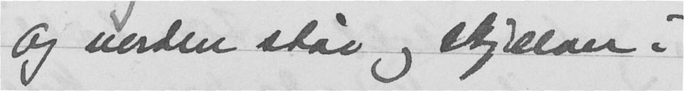Og verden står og skjelver:
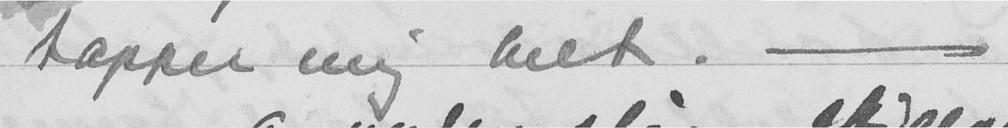tapper mig helt. -
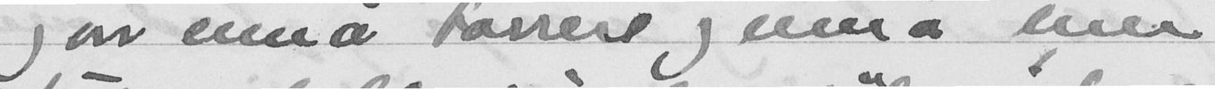og var ennå tørrere og ennå mer
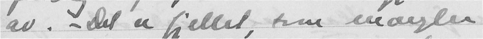av. - Det er fjellet, som mangler
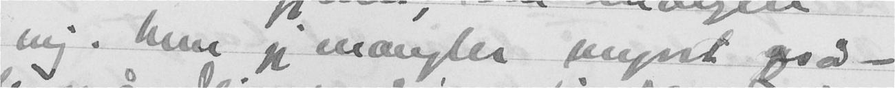mig. Men jeg mangler myrt ood -
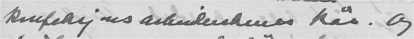konfeksjonarbeidernes kår. Og
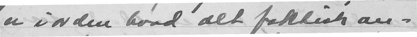er i orden hvad alt faktisk an-
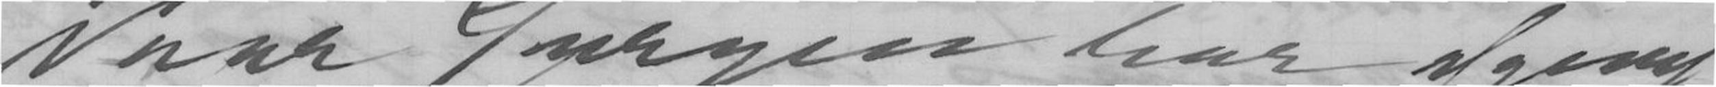Naar Juryen har afgivet
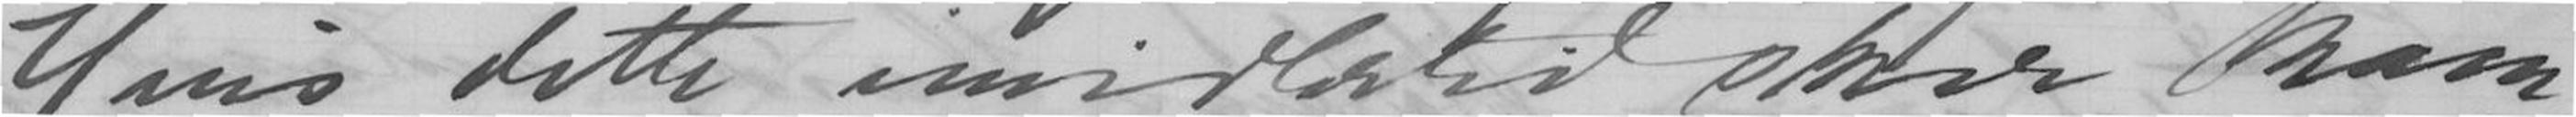Hvis dette imidlertid sker kom
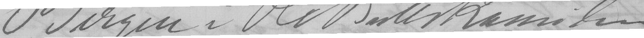Bergen i Ole Bull Komiteen
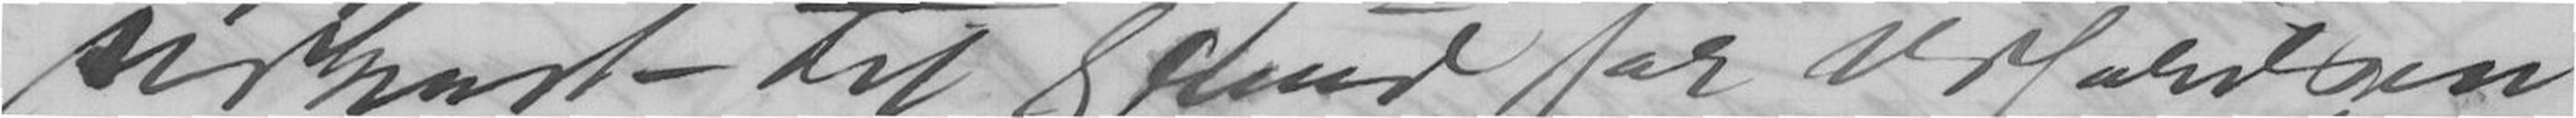Udkast til Grund for Udførelsen.
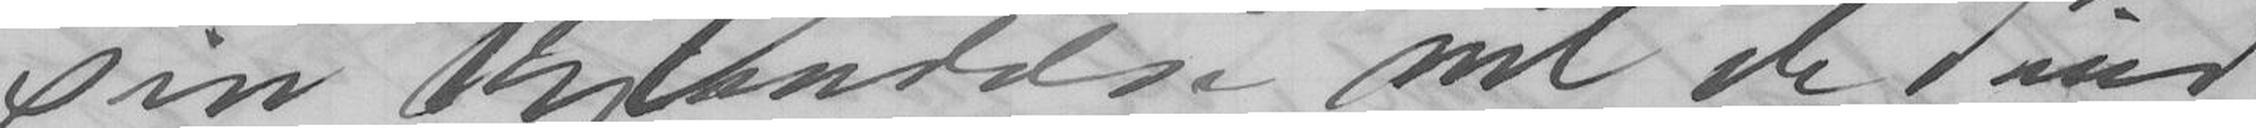sin Kjændelse vil de ind-
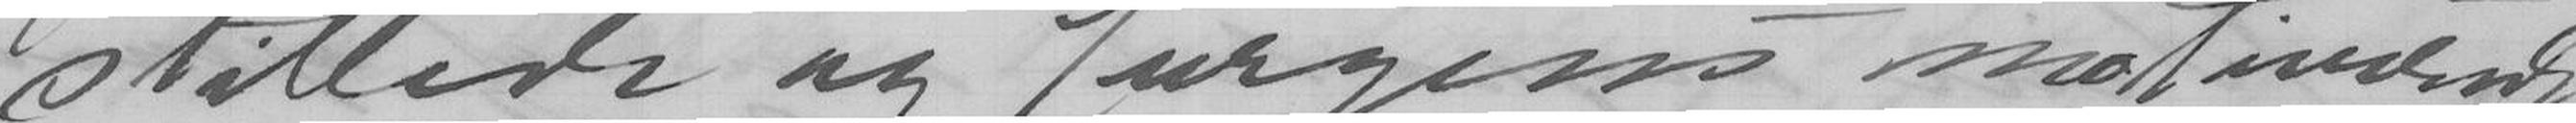stillede og Juryens motiværede
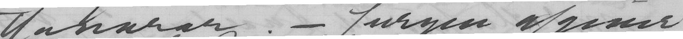Honorar. - Juryen afgiver
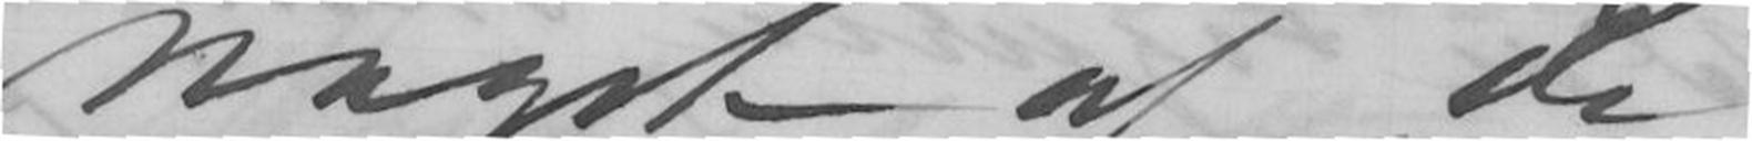noget af de
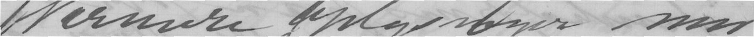Nærmere oplysninger med-
